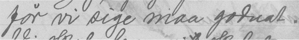før vi sige maa godnat.
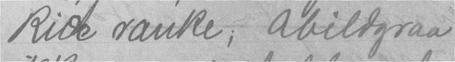Ride ranke; abildgraa
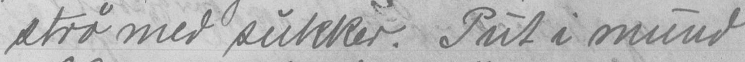strø med sukker. Put i mund
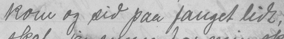kom og sid paa fanget lidt,
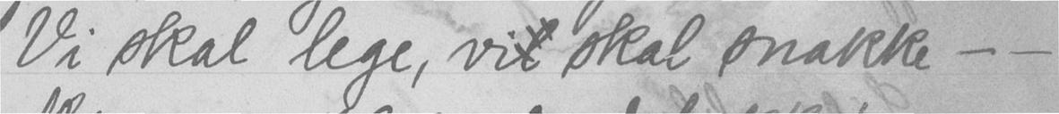Vi skal lege, vil skal snakke - -
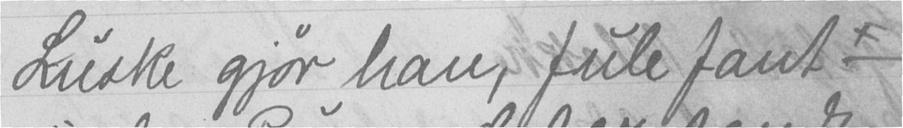Luske gjør han, fule fant -
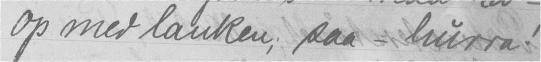op med lanken, saa - hurra!
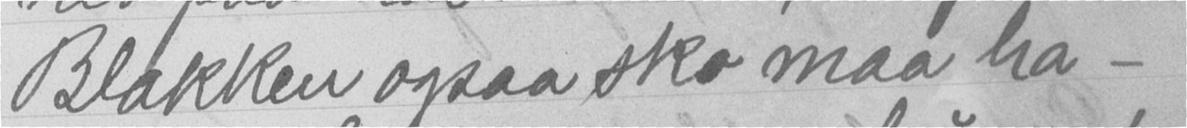Blakken ogsaa sko maa ha -
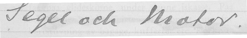Segel och Motor
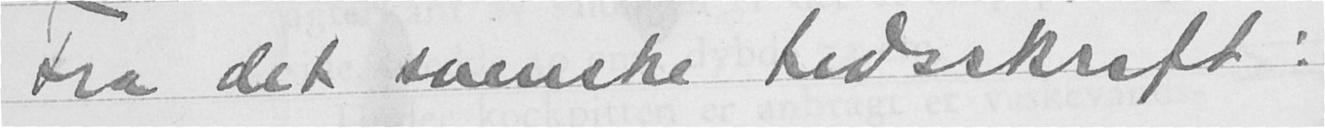Fra det svenske tidsskrift:
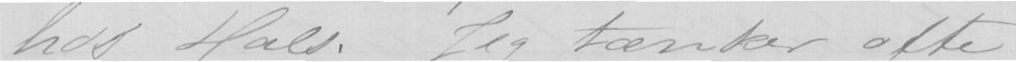hos Hals. Jeg tænker ofte
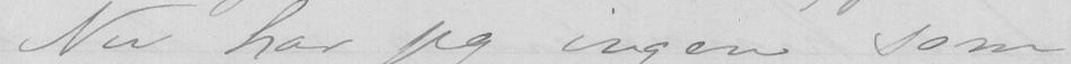Nu har jeg ingen som
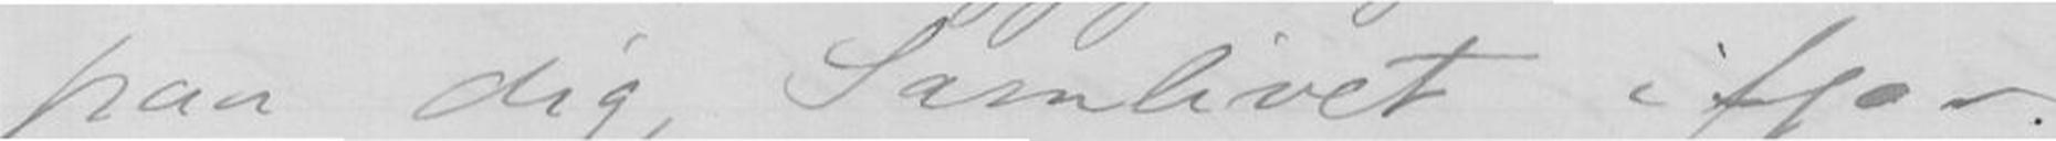paa dig, Samlivet ifjor.
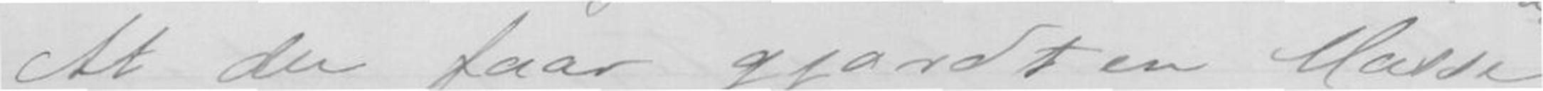At du faar gjordt en Masse
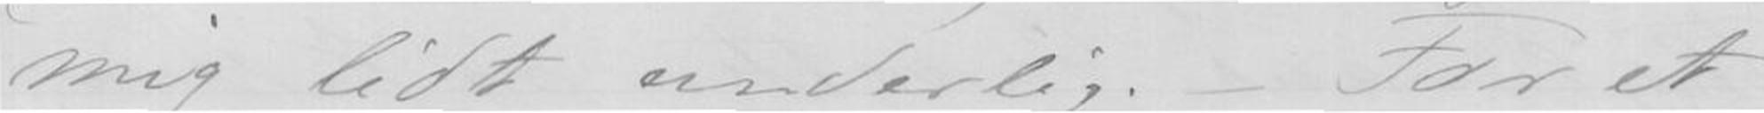mig lidt underlig. - For et
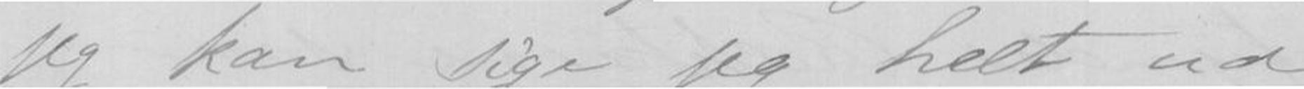jeg kan sige jeg helt ud
